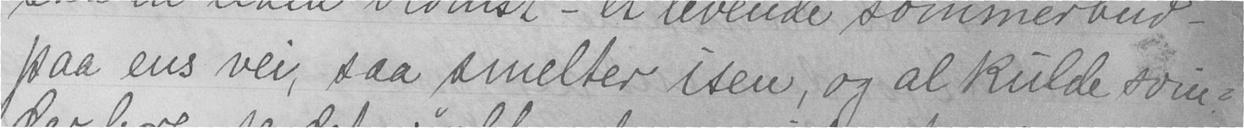paa ens vei, saa smelter isen, og al kulde svin-
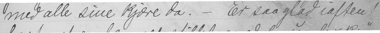med alle sine kjære da. - Er saa glad iaften!
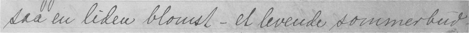saa en liden blomst - et levende sommerbud -
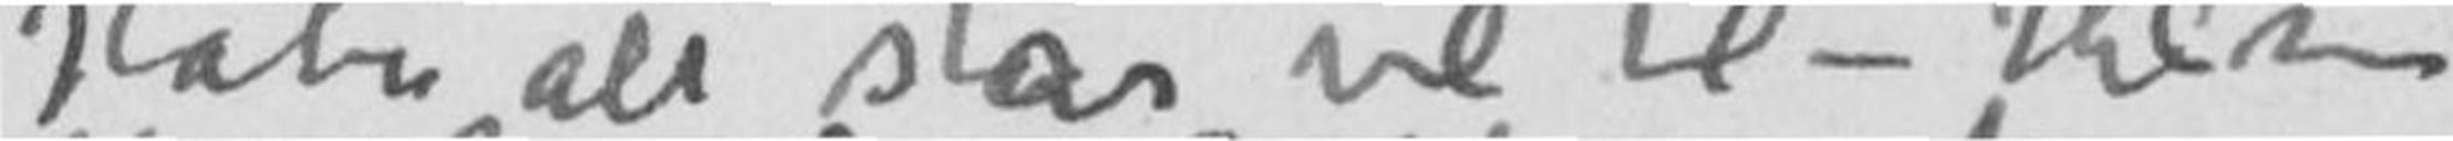Håber alt står vel til – Hilsen
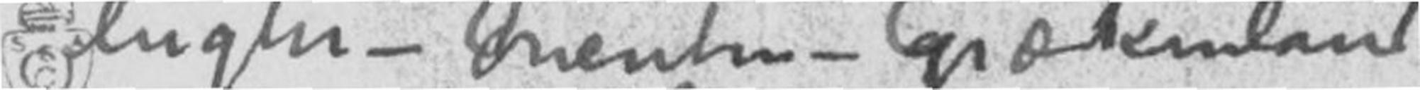lugter – Orienten – Grækenland
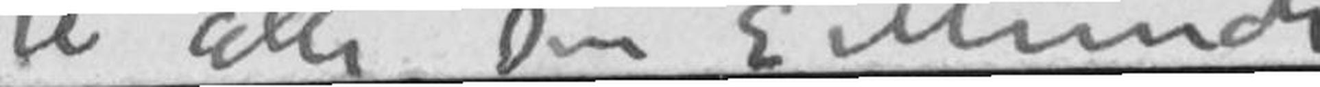til alle Din E Munch
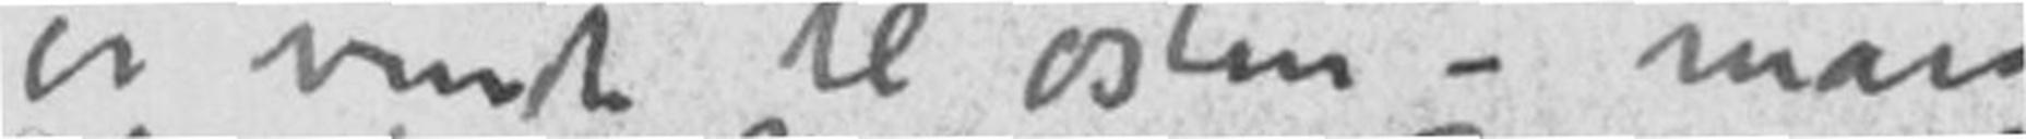er vendt til Østen – man
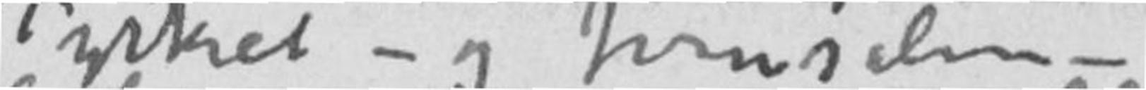Tyrkiet – og Jerusalem –
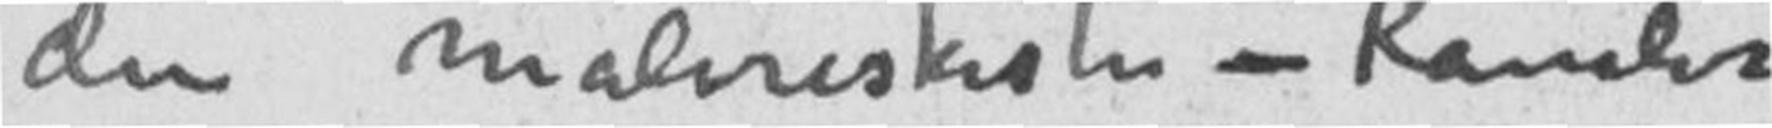den maleriskeste – Kanaler
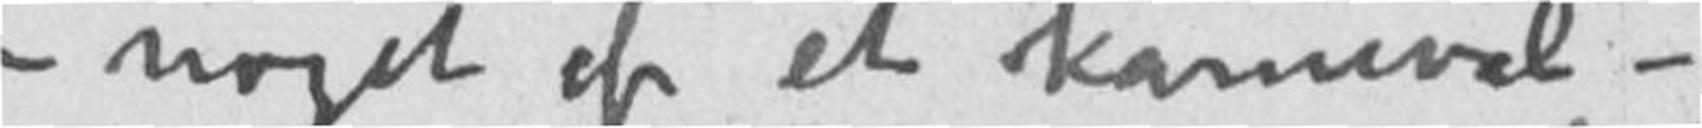– noget af et Karneval –
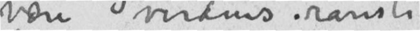være verdens rareste
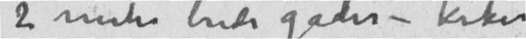2 meter brede gader – Kirker
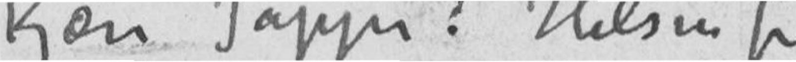Kjære Jappe! Hilsen fra
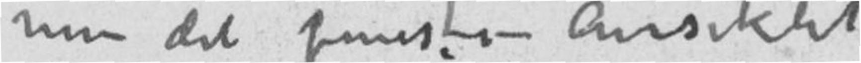men det fineste – Ansigtet
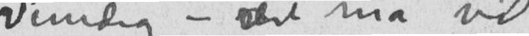Venedig – det må vel
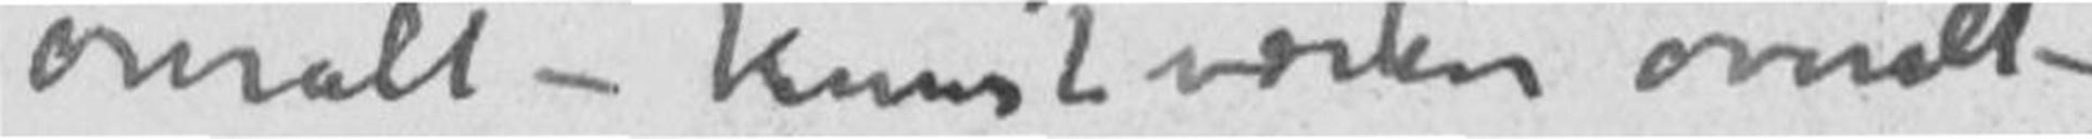overalt – Kunstværker overalt –
